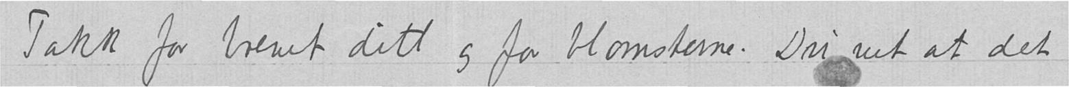Takk for brevet ditt og for blomsterne. Du vet at det
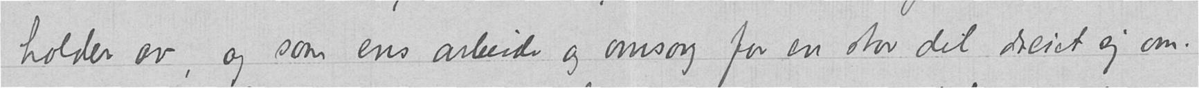holder av, og som ens arbeide og omsorg for en stor del dreiet sig om.
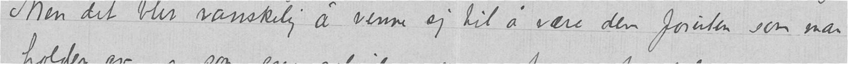Men det blir vanskelig å venne sig til å være dem foruten som man
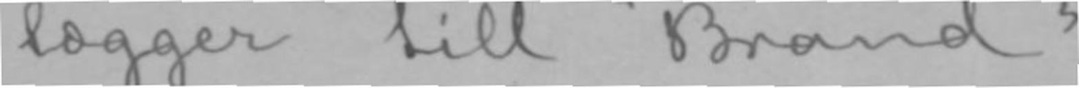lægger till «Brand».
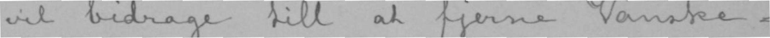vil bidrage till at fjerne Vanske-
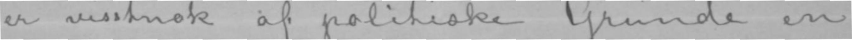er visstnok af politiske Grunde en
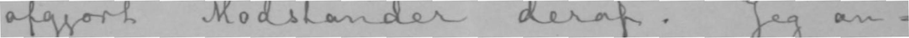afgjort Modstander deraf. Jeg an-
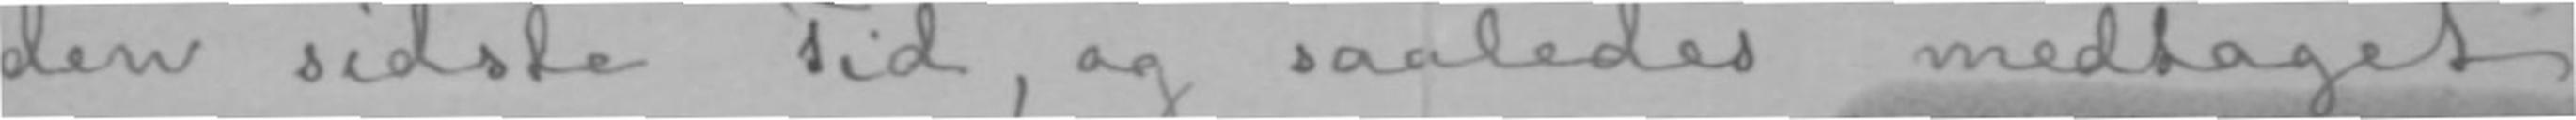den sidste Tid, og saaledes medtaget
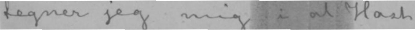tegner jeg mig i al Hast
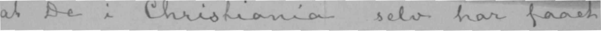at De i Christiania selv har faaet
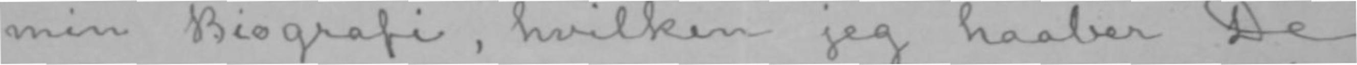min Biografi, hvilken jeg haaber De
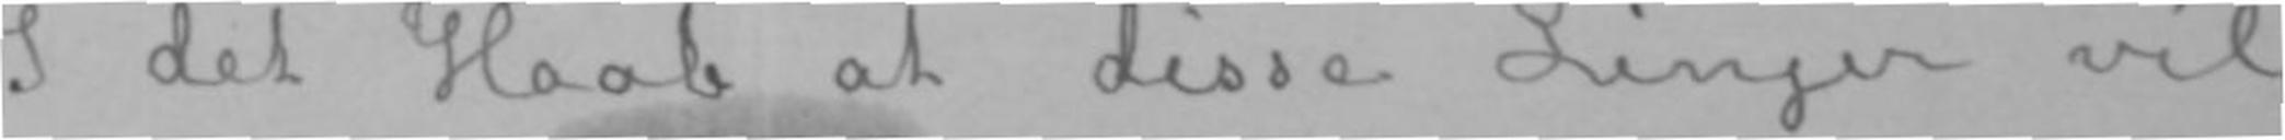I det Haab at disse Linjer vil
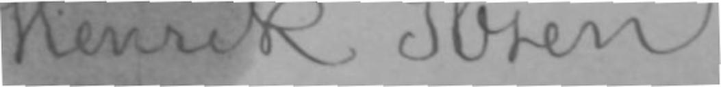Henrik Ibsen.
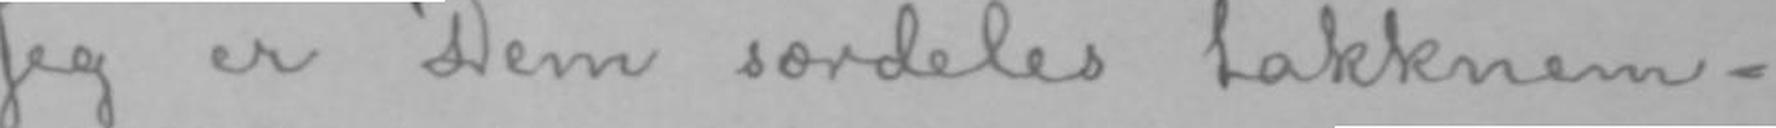Jeg er Dem særdeles takknem-
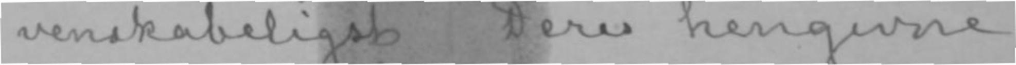venskabeligst Deres hengivne
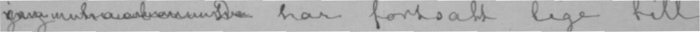jeg haaber De har fortsatt lige till
